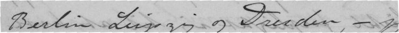Berlin, Leipzig og Dresden, - jeg
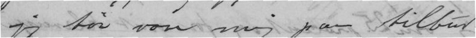jeg tør vove mig paa tilbud
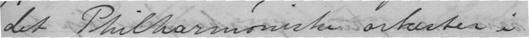det Philharmoniske orkester i
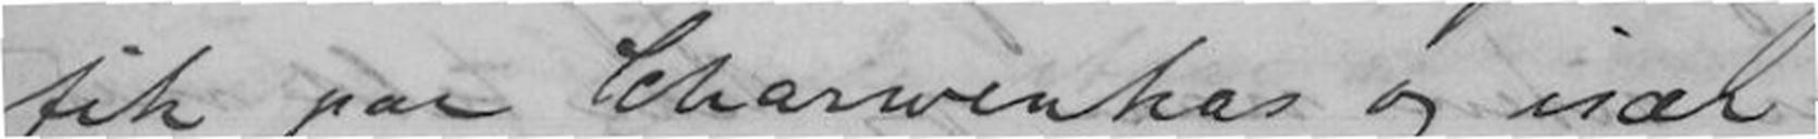fik paa Scharwenkas og især
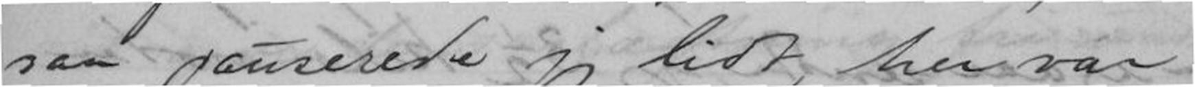saa pauserede jeg lidt, her var
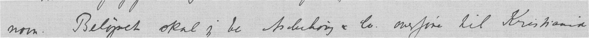navn. Beløpet skal jeg be Aschehoug & Co. overføre til Kristiania
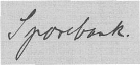Sparebank.
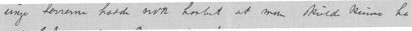unge herrerne hadde nok haabet at man skulde kunne ha
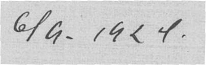6/9-1924.
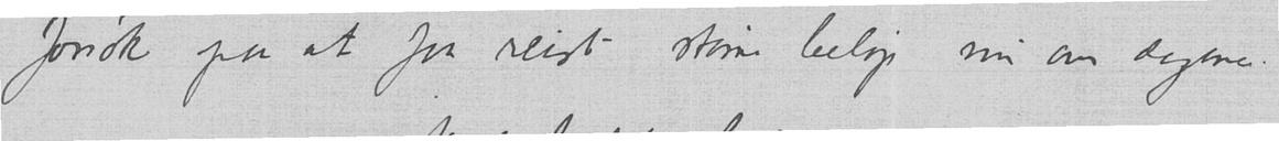forsøk paa at faa reist større beløp nu om dagene.
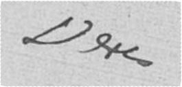Deres
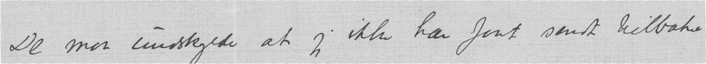De maa undskylde at jeg ikke har faat sendt tilbake
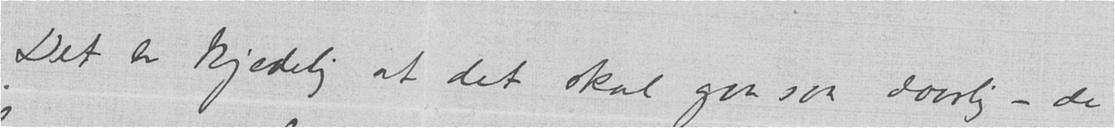Det er kjedelig at det skal gaa saa daarlig - de
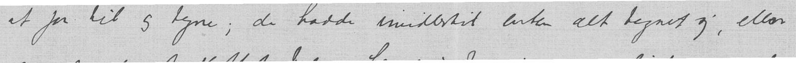at faa til og tegne; de hadde imidlertid enten alt tegnet sig, eller
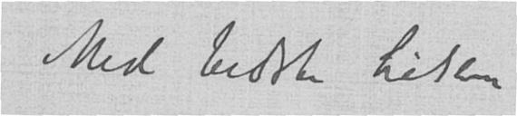Med bedste hilsen
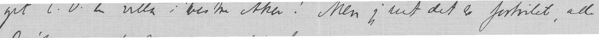git C.V. en villa i Vestre Aker! Men jeg vet det er fortvilet, alle
